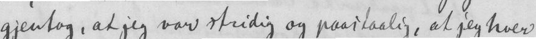gjentog, at jeg var stridig og paastaalig, at jeg hver-
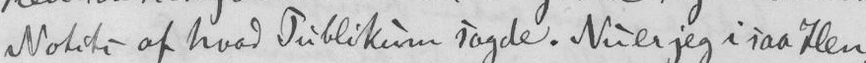Notits af hvad Publikum sagde. Nu er jeg i saa Hen-
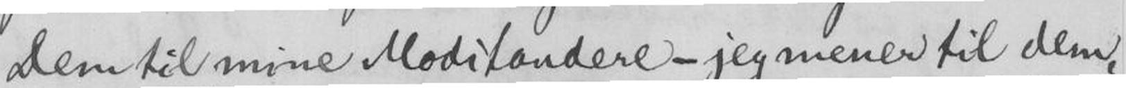Dem til mine Modstandere - jeg mener til dem,
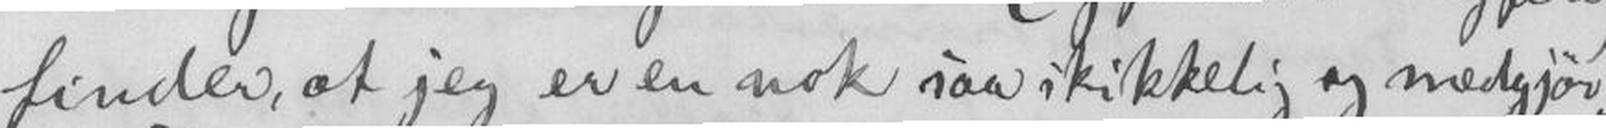finder, at jeg er en nok saa skikkelig og medgjør-
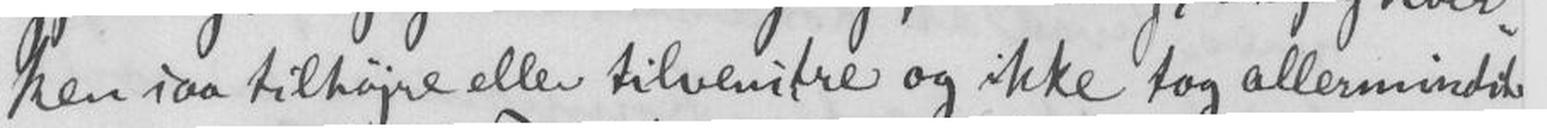ken saa tilbage eller tilhøjre eller tilvenstre og ikke tog allemindste
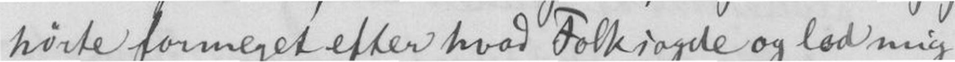hørte formeget efter hvad Folk sagde og lod mig
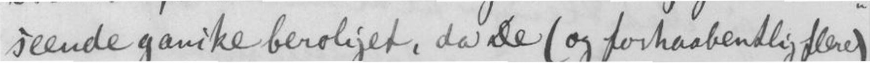seende ganske beroliget, da De (og forhaabentlig flere)
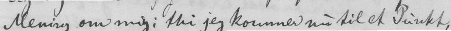Mening om mig; thi jeg kommer nu til et Punkt,
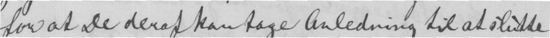for at De deraf kan tage Anledning til at Slutte
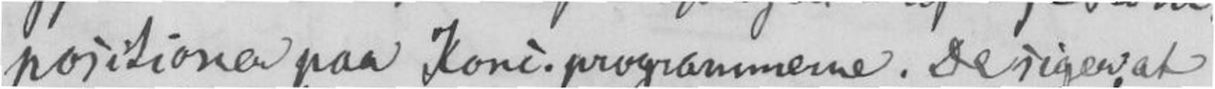posioner paa Kons.programmerne. De siger at
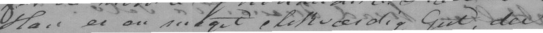Han er en meget elskværdig Gut, der
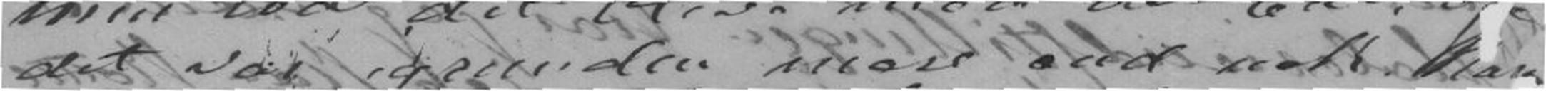det var grunden mere end nok. Han
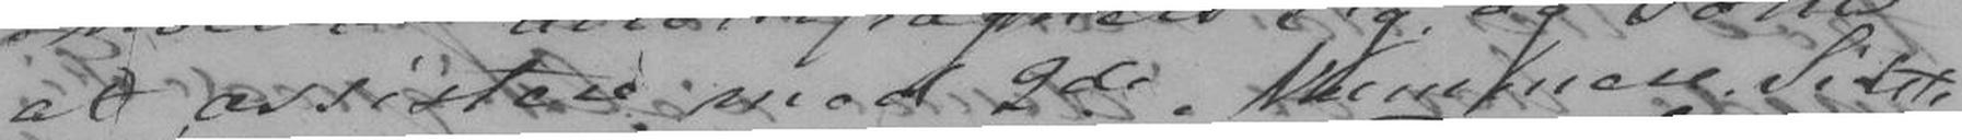at assistere med 2de Nummere Sidste
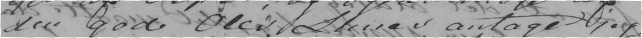den gode Oles Luner antage jeg.
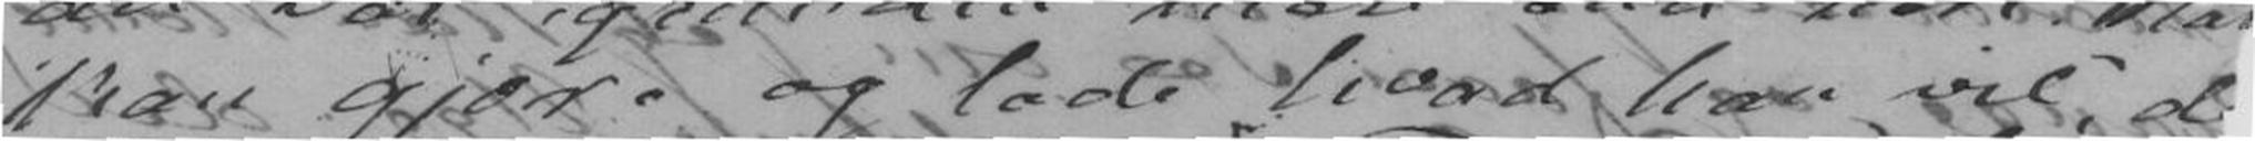kan gjøre og lade hvad han vil, der
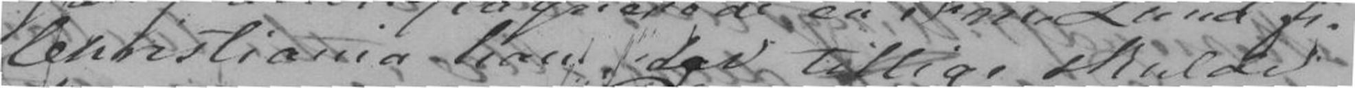Christiania han der tillige skulde
